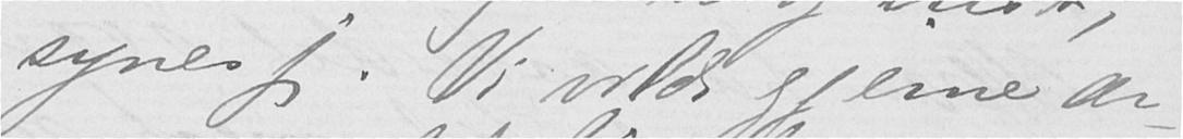synes jeg. Vi vilde gjerne ar-
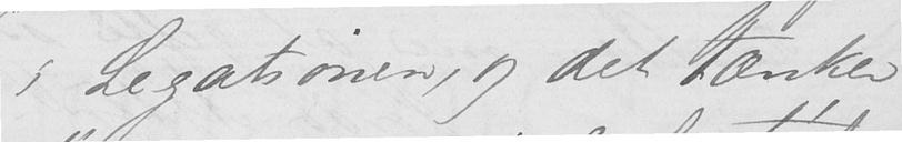i Legationen, og det tænker
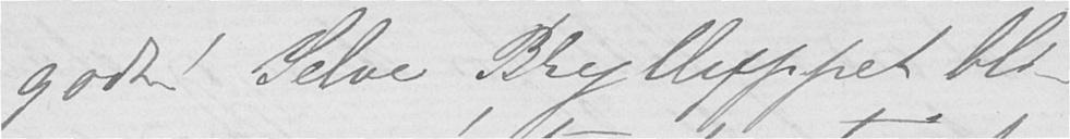godt! Selve Brylluppet bli-
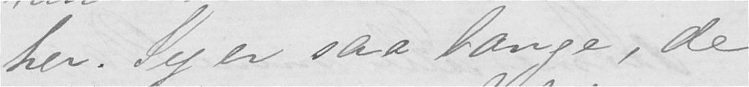her. Jeg er saa bange, de
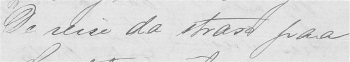De reise da strax paa
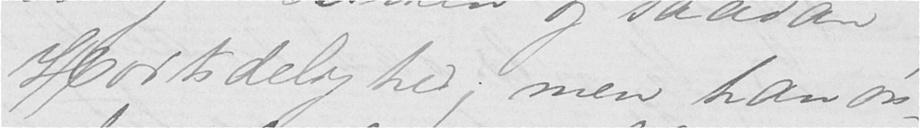Høitidelighed; men han øn-
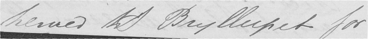herved til Bryllupet for
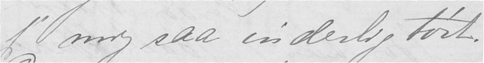jeg mig saa inderlig tørt.
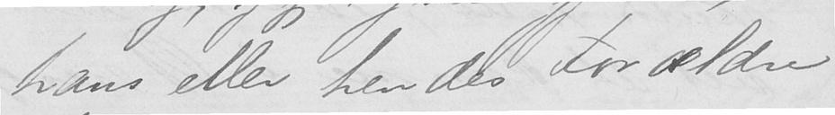hans eller hendes Forældre
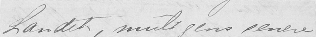Landet, muligens senere
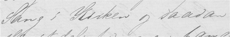Sang i Kirken og saadan
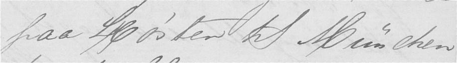paa Høsten til München,
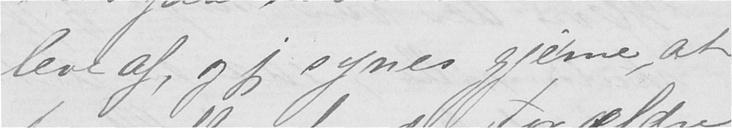leve af, og jeg synes gjerne, at
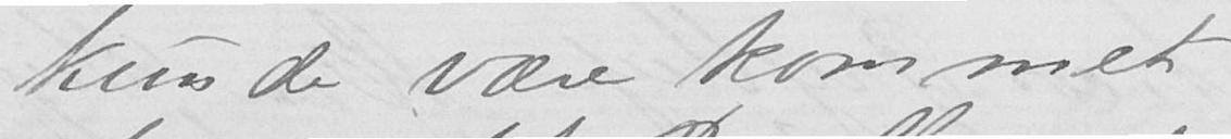kunde være kommet
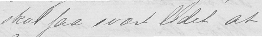skal faa svært lidet at
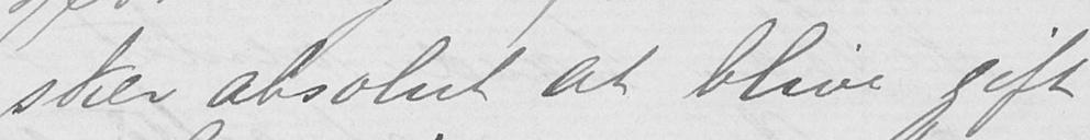sker absolut at blive gift
